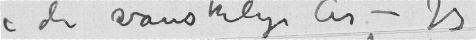i de vanskelige År – Jeg
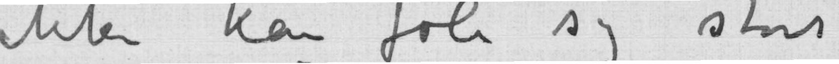ikke kan føle sig stort
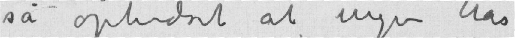så ophidset at ingen har
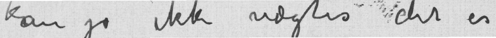kan jo ikke nægtes det er
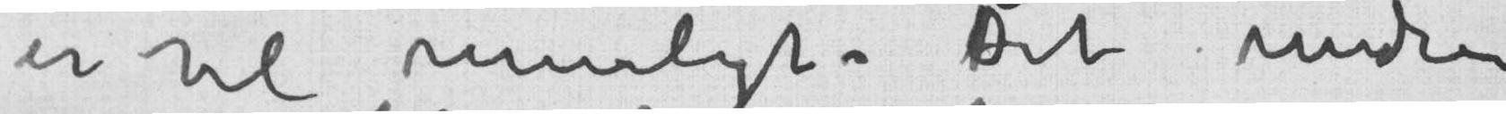er vel muligt – Det undrer
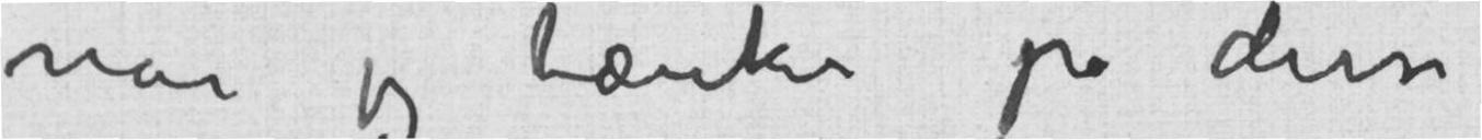når jeg tænker på disse
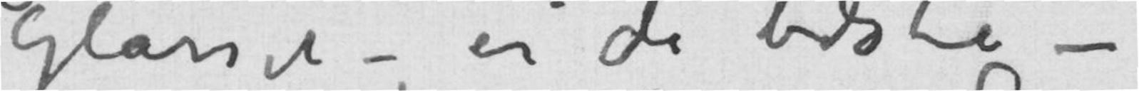Glasset – er de bedste –
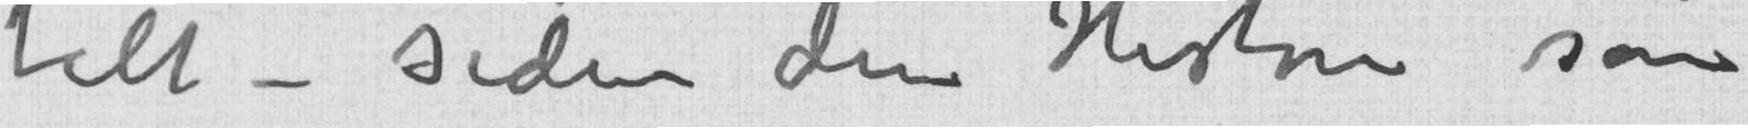talt – siden den Historie som
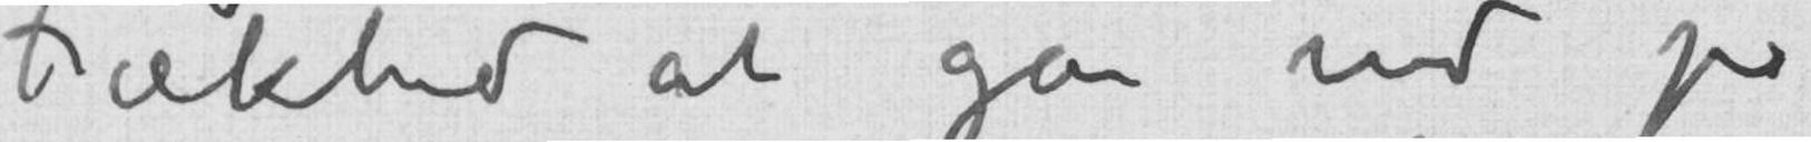Frækhed at gå ind på
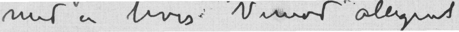med en hvis Vemod alligevel
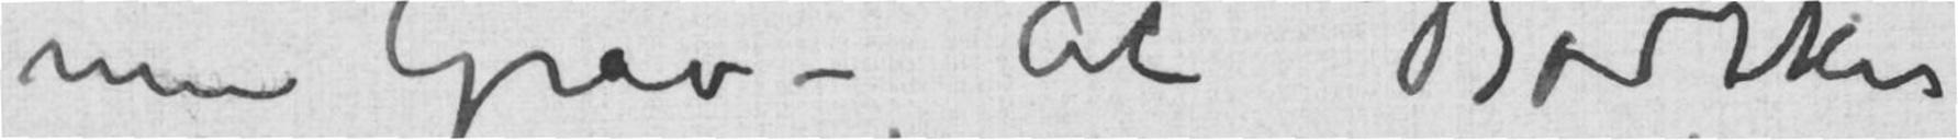min Grav – At Bødtker
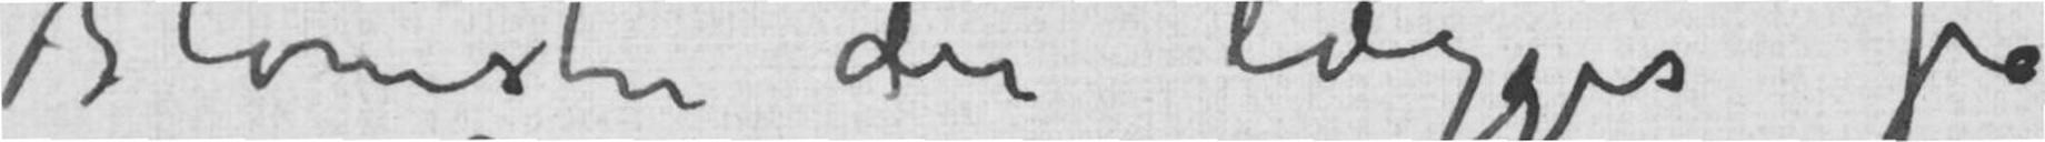Blomster der lægges på
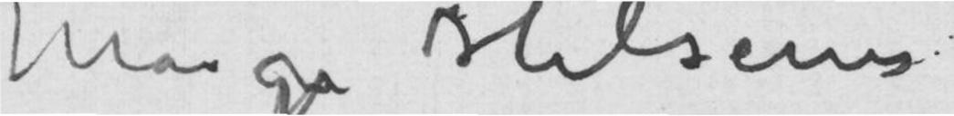Mange Hilsener
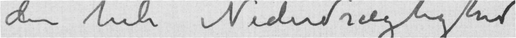den hele Nederdrægtighed
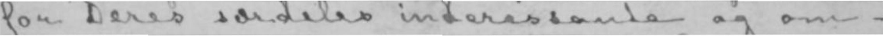for Deres særdeles interessante og om-
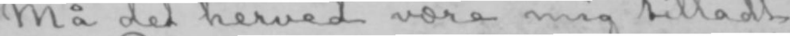Må det herved være mig tilladt
